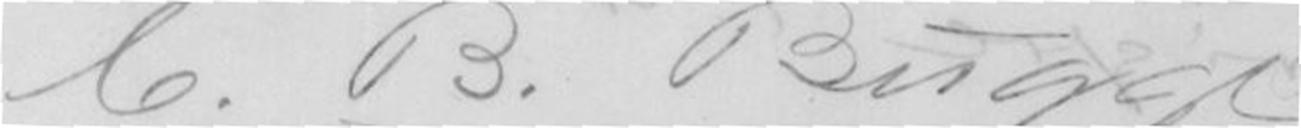C. B. Bugge.
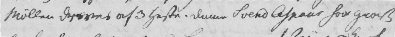Møllen drives af 3 Heste. Denne Svend Aspaas har giort
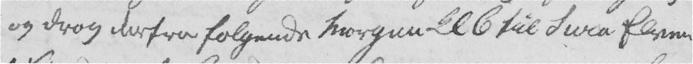og drog derfra følgende Morgen Kl 6 til Sura Elven
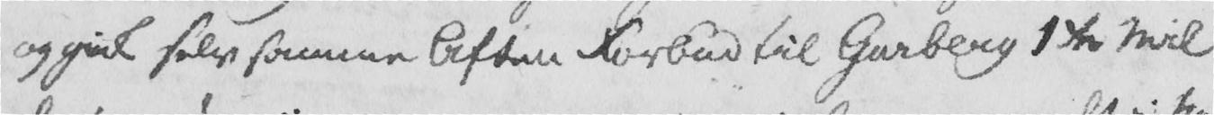og gik selv samme Aften Forbud til Garberg 1 1/2 Mil
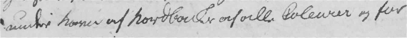under Navn af Nordlandske af alle Coleurer og for
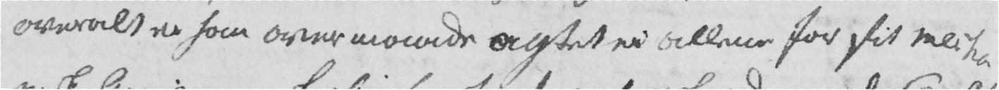Overalt er han overmaade agtet ei allene for sit mecka-
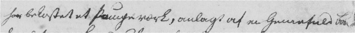har bekostet et Pumpeværk, anlagt af en Geniefuld Bon-
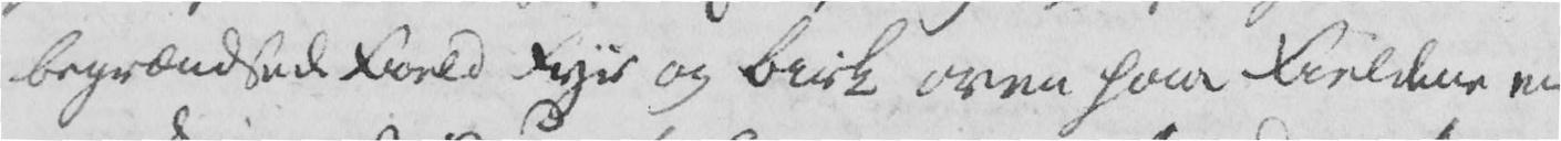begrændsede Field Fyr og Birk oven paa Fieldene er
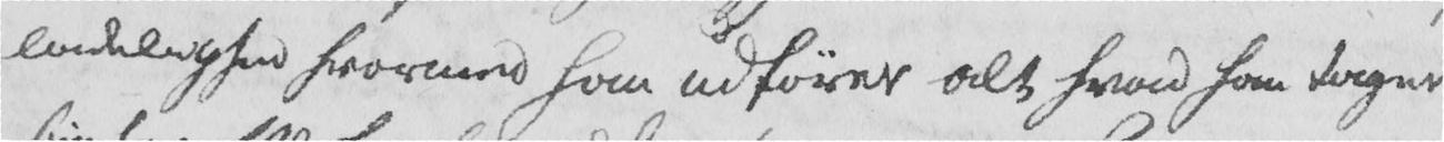ladelighed hvormed han udfører alt hvad han tager
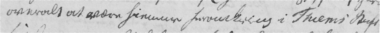overalt at være hiemme heromkring i Thiems Stift.
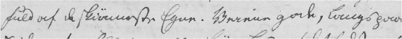fuld af de skiønneste Egne. Veiene gode, langs paa
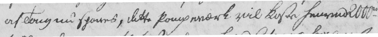af Taug nu spares, dette Pompeværk vil koste henved 2000 rd -
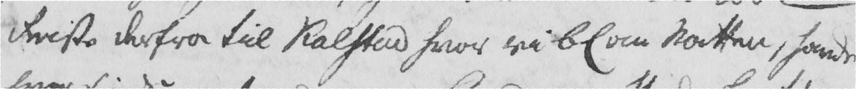Reiste derfra til Kalstad hvor vi bl om Natten, havde
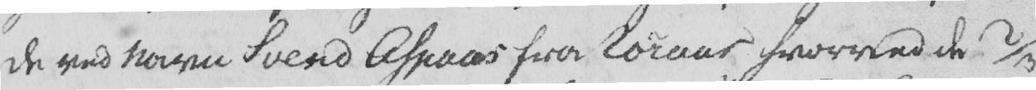de ved Navn Svend Aspaas fra Røraas hvorved de 2/3
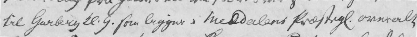til Garberg Kl. 9. som ligger i Meldalens Præstegl. overalt
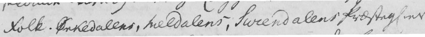Folk. Ørkedalens, Meldalens, Surendalens Præstegl ere
